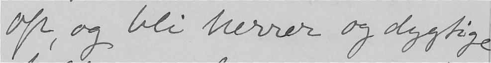op, og bli herrer og dygtige
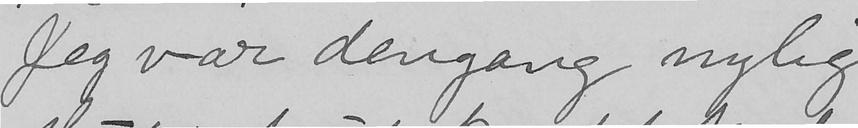Jeg var dengang nylig
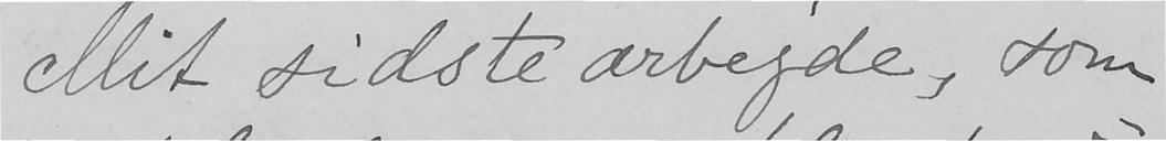Mit sidste arbejde, som
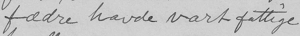fædre havde vært fattige
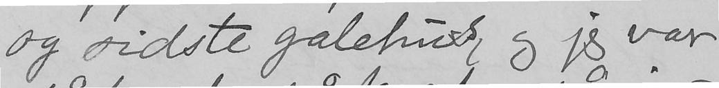og sidste galehus, og jeg var
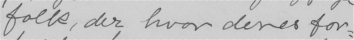folk, der hvor deres for-
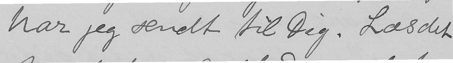har jeg sendt til Dig. Læs det
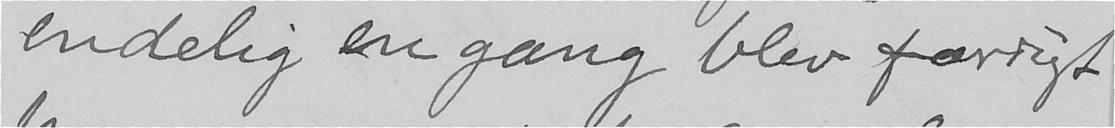endelig en gang blev færdigt
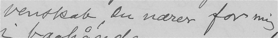venskab, Du nærer for mig,
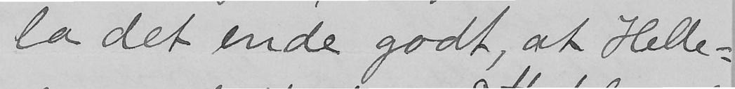la det ende godt, at Helle-
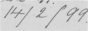14/2/99.
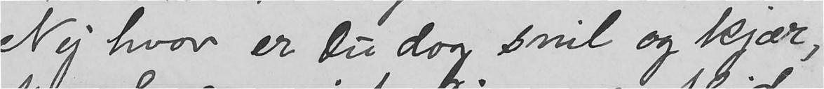Nei hvor er Du dog snil og kjær,
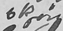skjøn
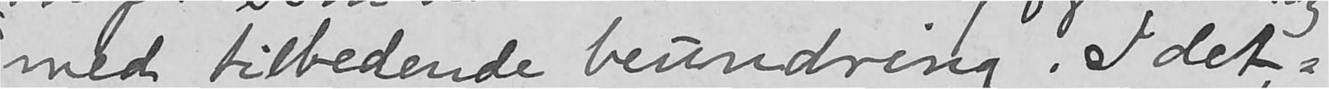med tilbedende beundring. Idet-
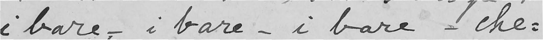i bare, - i bare - i bare - che-
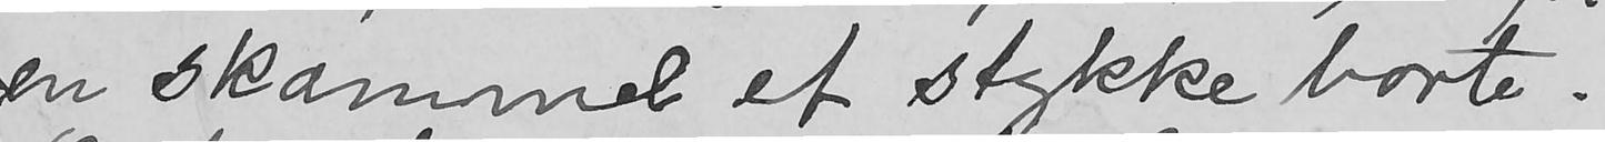en skammel et stykke borte.
